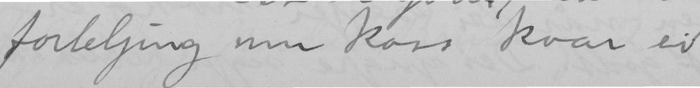forteljing um koss kvar ei
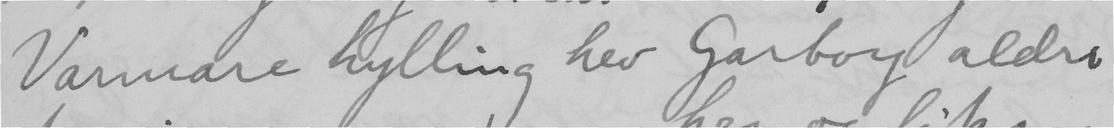Varmare hylling hev Garborg aldri
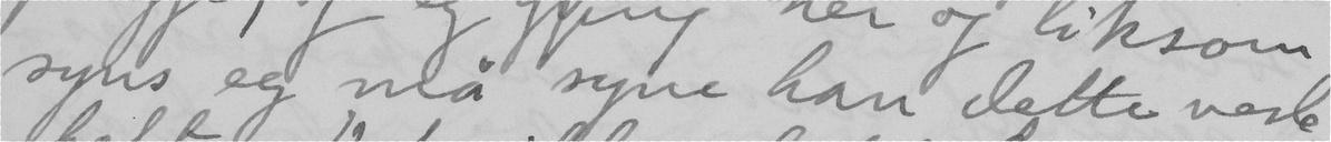syns eg må syne han dette vesle
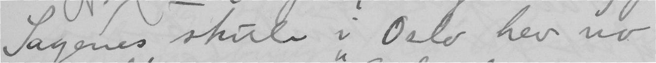Sagenes skule i Oslo hev no
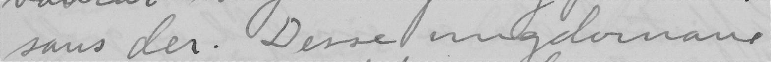sans der. Desse ungdomane
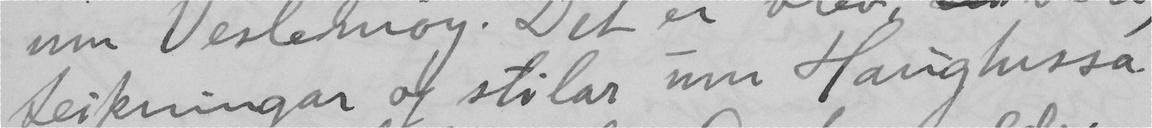teikningar og stilar um Haugtussa.
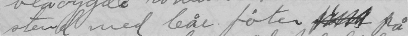stend med båe föter  på
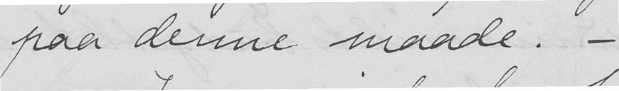paa denne maade. -
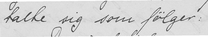talte sig som følger:
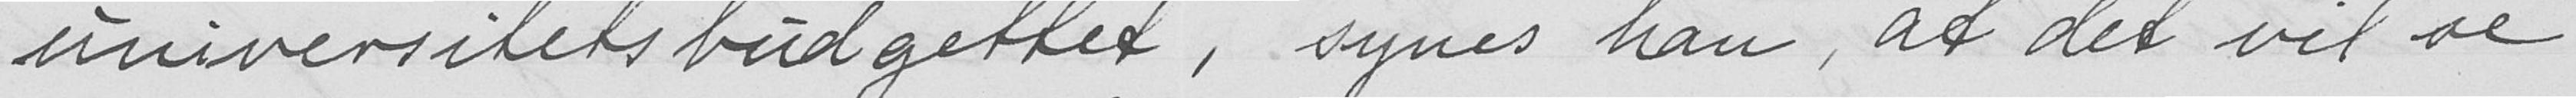universitetsbudgettet, synes han, at det vil se
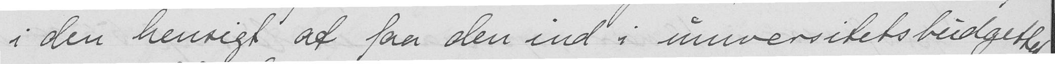i den hensigt at faa den ind i universitetsbudgettet,
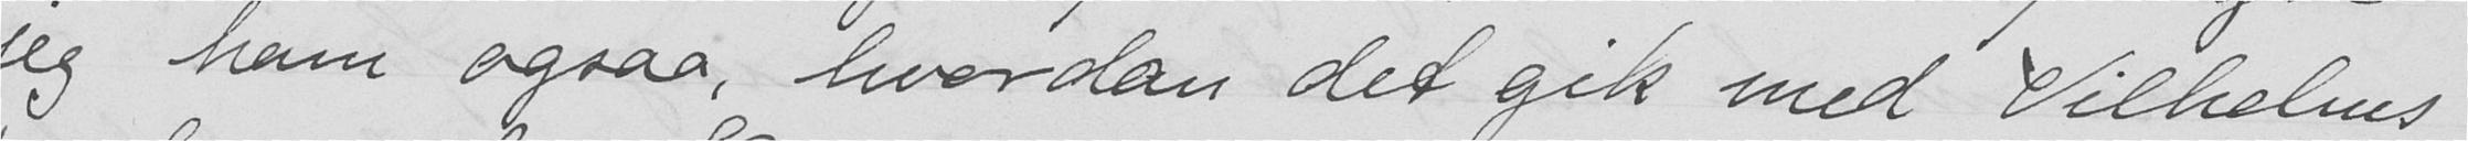jeg ham ogsaa, hvordan det gik med Vilhelms
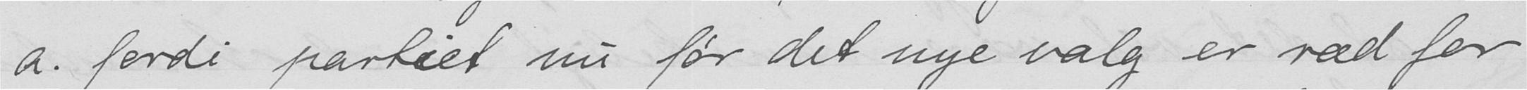a. fordi partiet nu før det nye valg er ræd for
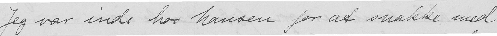Jeg var inde hos Nansen for at snakke med
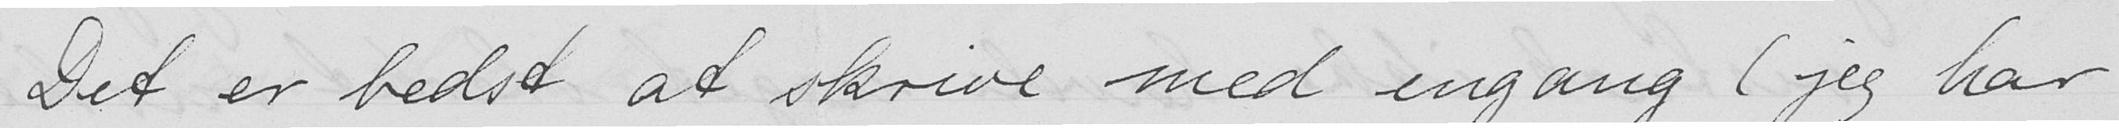Det er bedst at skrive med engang (jeg har
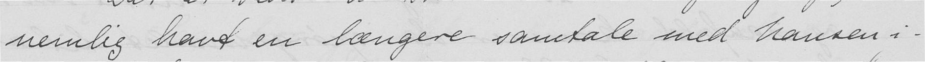nemlig havt en længere samtale med Nansen i-
